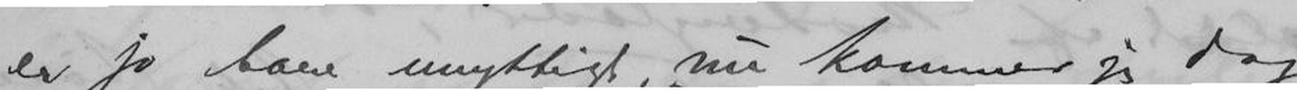er jo bare unyttigt, nu kommer jeg dog
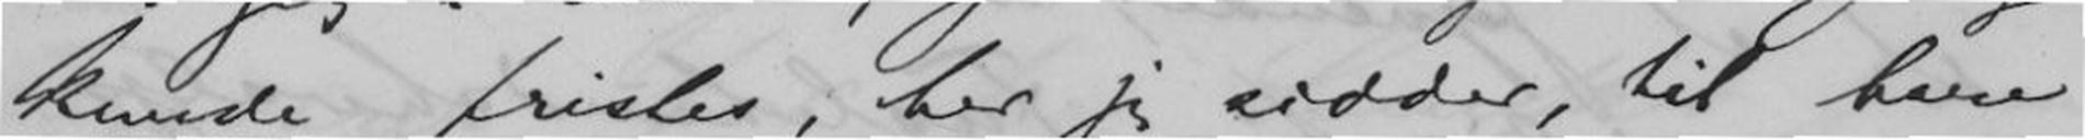kunde fristes; her jeg sidder, til bare
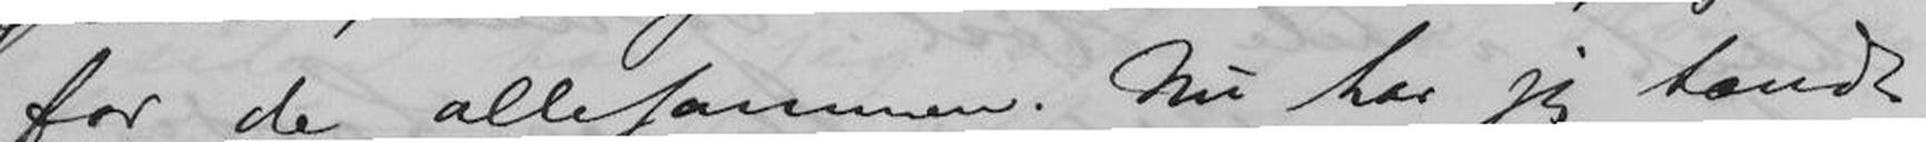for de allesammen. Nu har jeg tændt
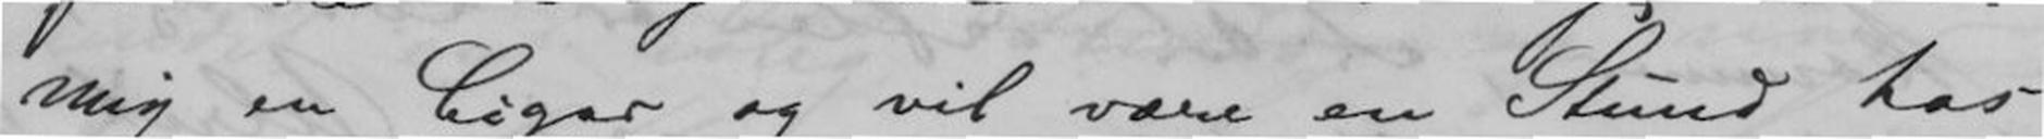mig en Cigar og vil være en Stund hos
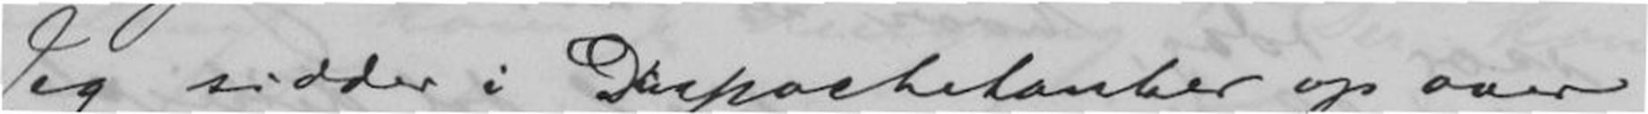Jeg sidder i Dispachetanker op over
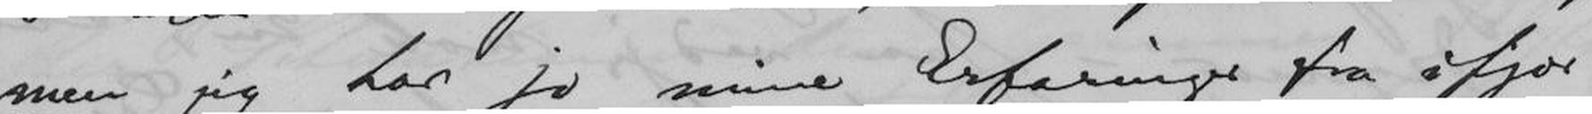men jeg har jo mine Erfaringer fra ifjor
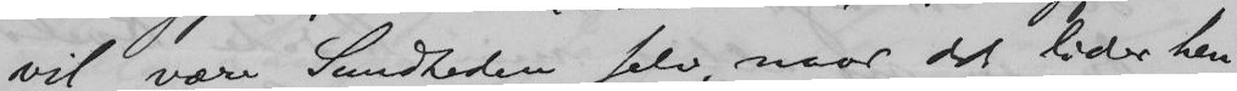vil være Sundheden selv, naar det lider hen
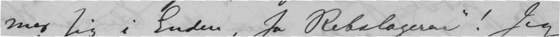mer sig i Enden, sa Rebslageren"! Jeg
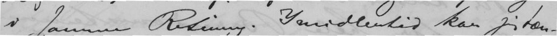i samme Retning. Imidlertid kan jeg tæn-
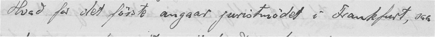Hvad for det første angaar juristmødet i Frankfurt, saa
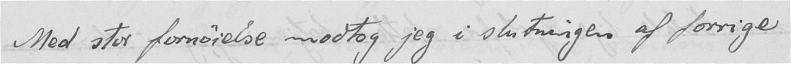Med stor fornøielse modtog jeg i slutningen af forrige
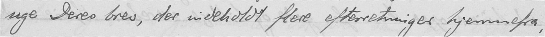uge Deres brev, der indeholdt flere efterretninger hjemmefra,
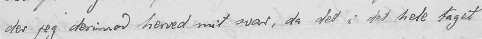der jeg derimod herved mit svar, da det i det hele taget
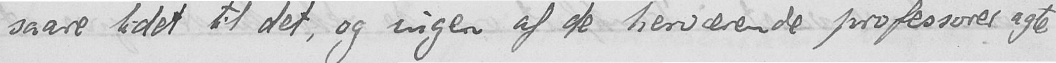saare lidet til det, og ingen af de herværende professorer agte
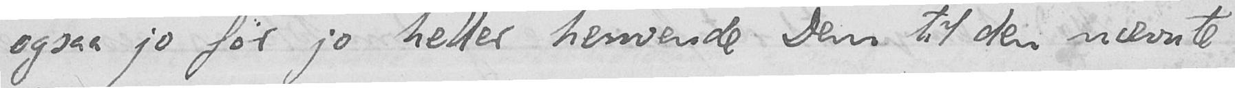ogsaa jo før jo heller henvende Dem til den nævnte
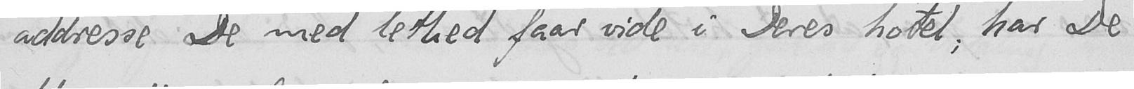addresse De med lethed faar vide i Deres hotel; har De
